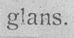glans.
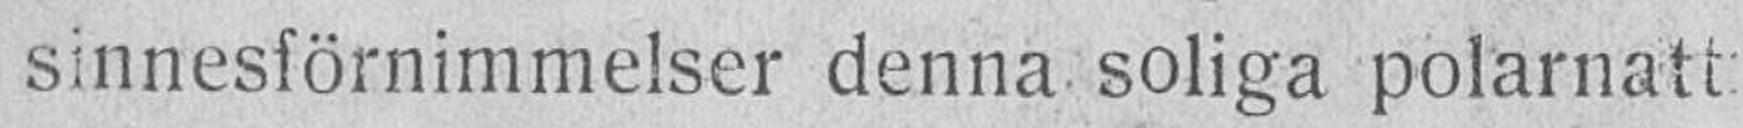sinnesförnimmelser denna soliga polarnatt
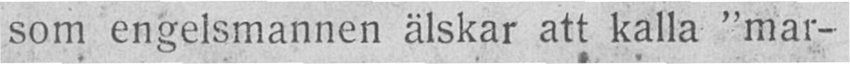som engelsmannen älskar att kalla "mar-
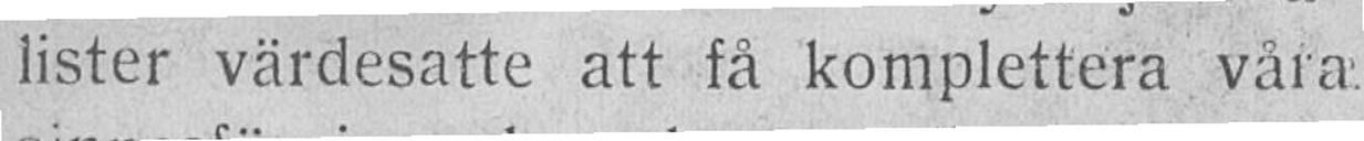lister värdesatte att få komplettera vära
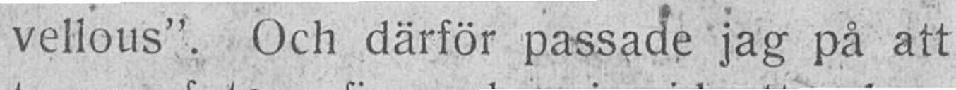vellous". Och därför passade jag på att
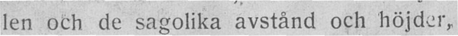len och de sagolika avstånd och höjder,
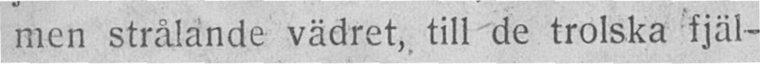men strälande vädret, till de trolska fjäl-
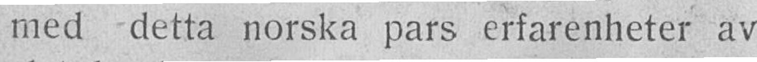med detta norska pars erfarenheter av
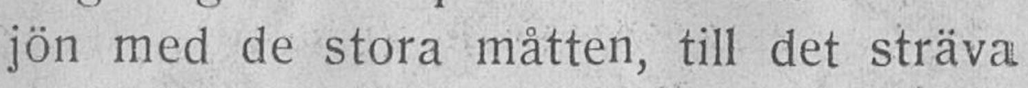jön med de stora måtten, till det sträva
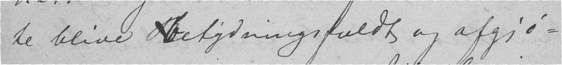te blive betydningsfuldt og afgjø-
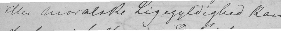eller moralske Ligegyldighed kan
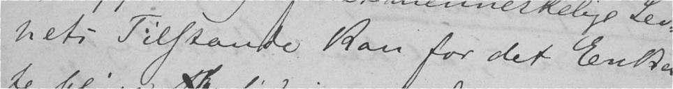nets Tilstande kan for det Enkel-
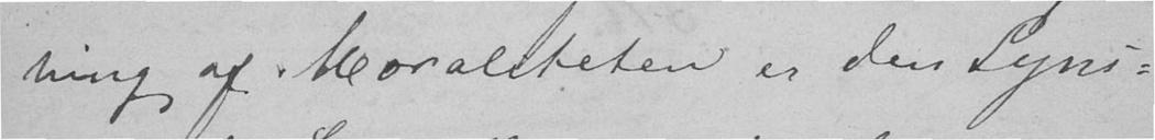ting af Moraliteten er den Syns-
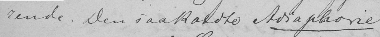rende. Den saakaldte Adiaphorie
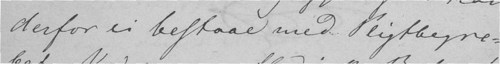derfor ei bestaae med Pligtbegre-
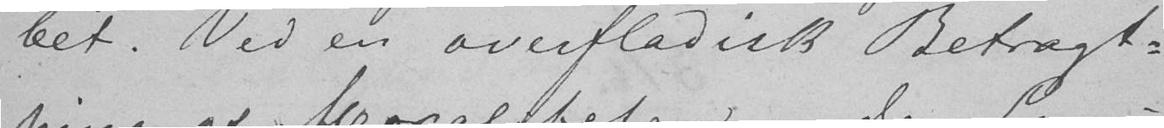bet. Ved en overfladisk Betragt-
